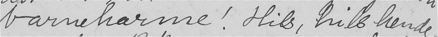barneharme! Hils, hils hende.
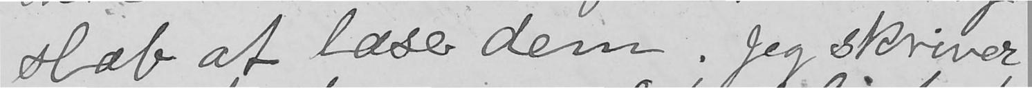slab at læse dem. Jeg skriver
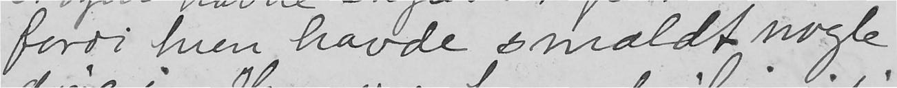fordi hun havde smældt nogle
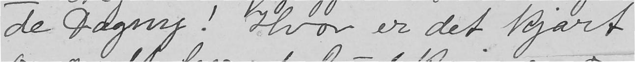de Dagny! Hvor er det kjært
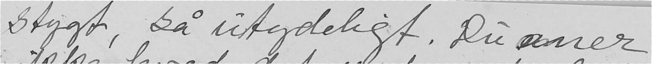stygt, så utydeligt, Du aner
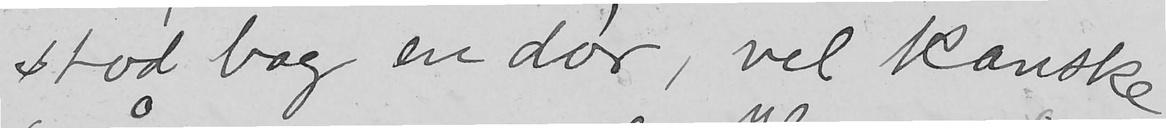stod bag en dør, vel kanske
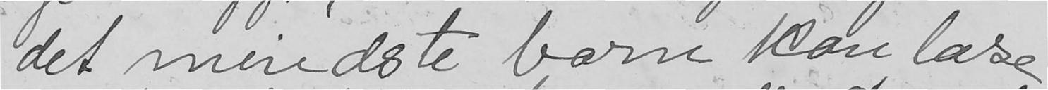det mindste barn kan læse
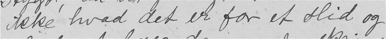ikke hvad det er for et slid og
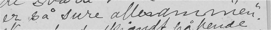er så sure allerammen".
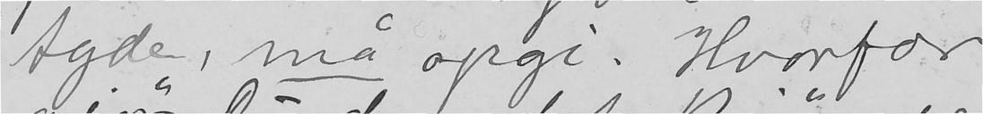tyde, må opgi. Hvorfor
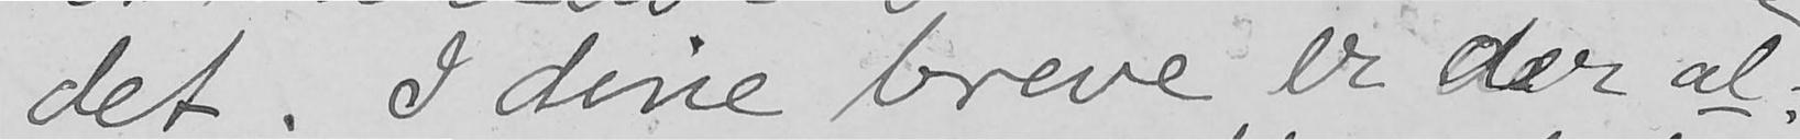det. I dine breve er der al-
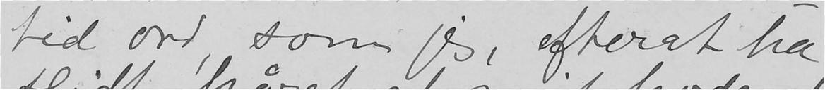tid ord, som jeg, efterat ha
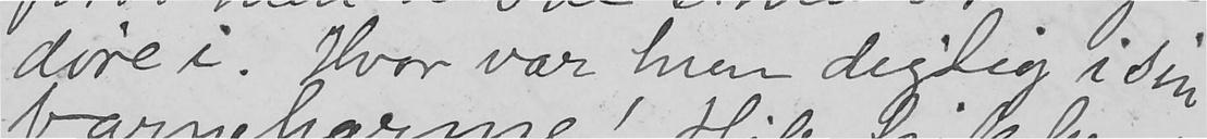døre i. Hvor var hun dejlig i sin
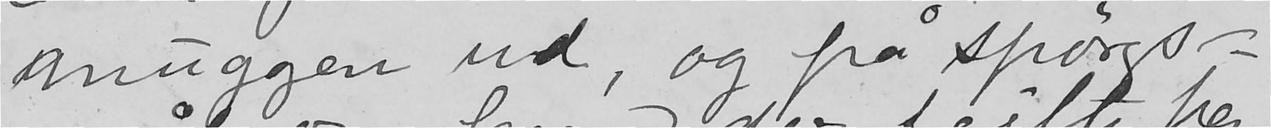muggen ud, og fra spørgs-
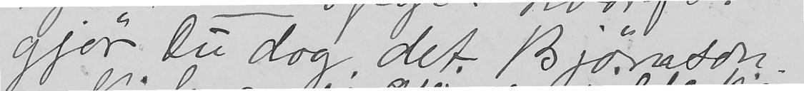gjør Du dog det Bjørnson.
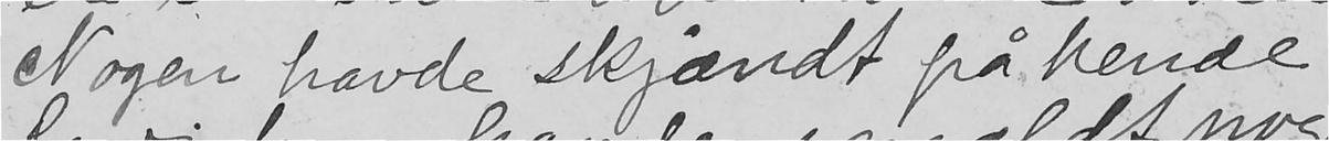Nogen havde skjændt på hende
